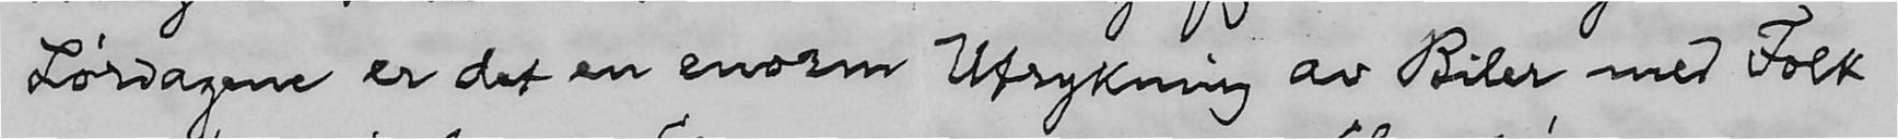Lørdagene er det en enorm Utrykning av Biler med Folk
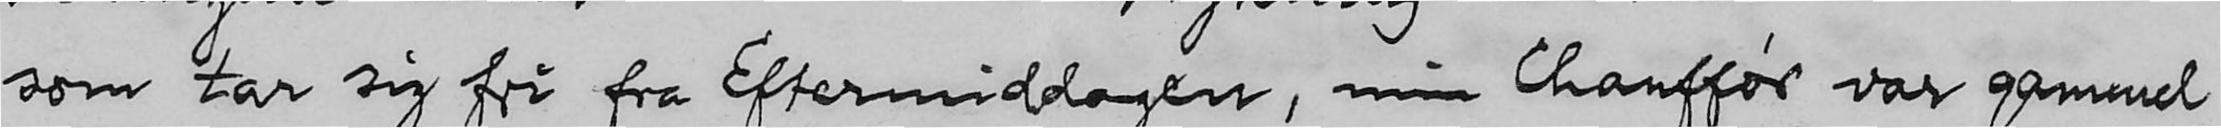som tar sig fri fra Eftermiddagen, min Chauffør var gammel
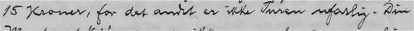15 Kroner, for det andet er ikke Turen ufarlig. Din
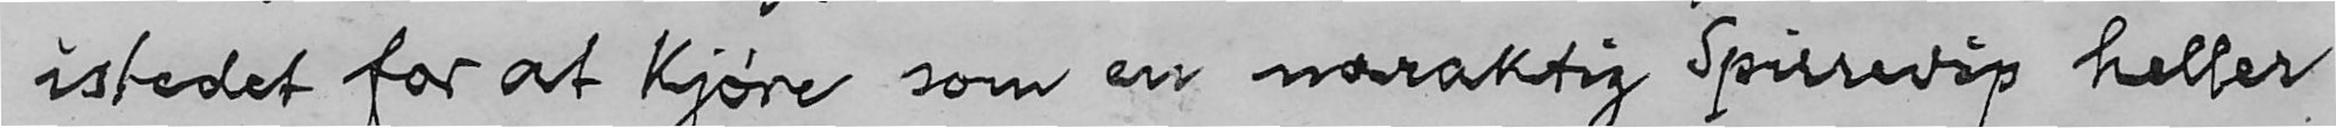istedet for at kjøre som en naraktig Spirrevip heller
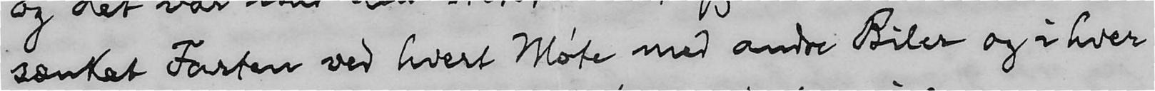sænket Farten ved hvert Møte med andre Biler og i hver
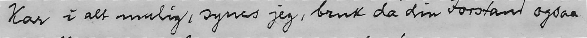Kar i alt mulig, synes jeg, bruk da din Forstand ogsaa
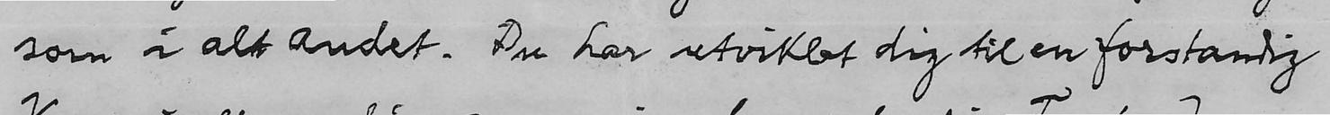som i alt andet. Du har utviklet dig til en forstandig
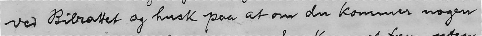ved Bilrattet og husk paa at om du kommer nogen
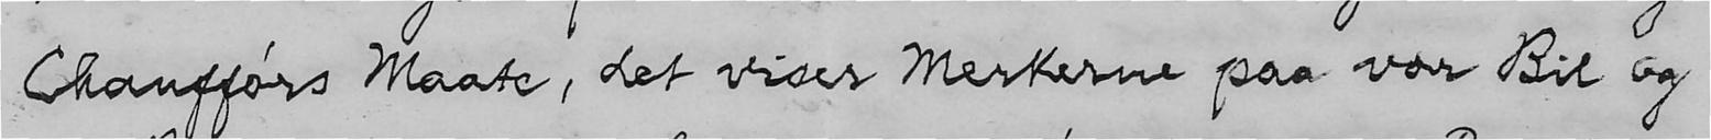Chaufførs Maate, det viser Merkerne paa vor Bil og
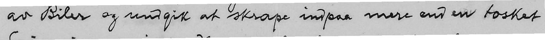av Biler og undgik at skrape indpaa mere end en tosket
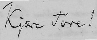Kjære Tore!
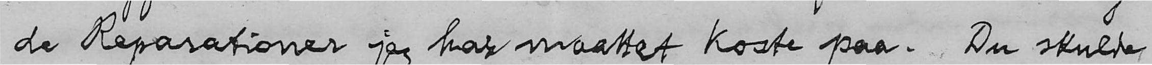de Reparationer jeg har maattet koste paa. Du skulde
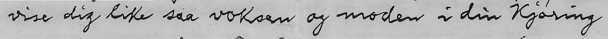vise dig like saa voksen og moden i din Kjøring
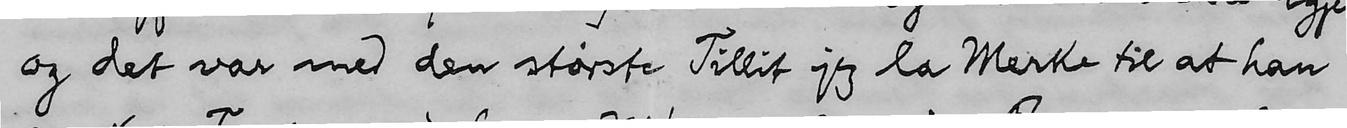og det var med den største Tillit jeg la Merke til at han
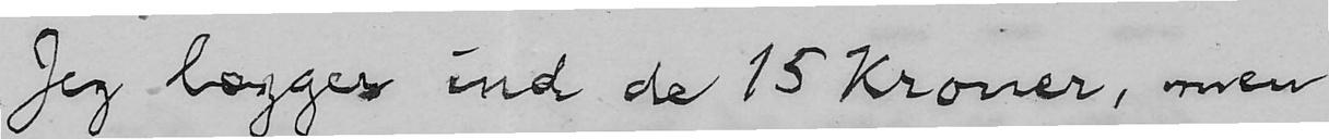Jeg lægger ind de 15 Kroner, men
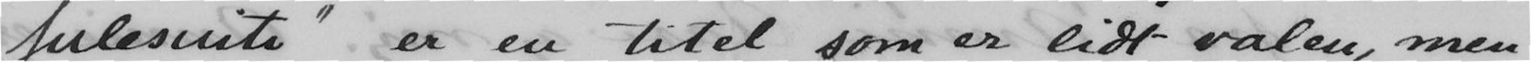"Julesuite" er en titel som er lidt valen, men
